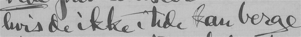hvis de ikke i tide kan berge
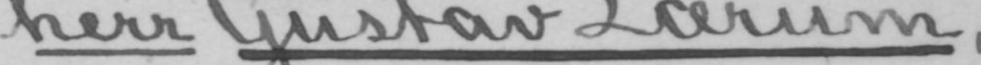herr Gustav Lærum,
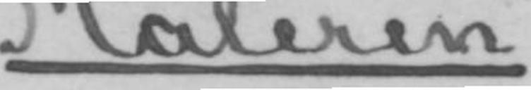Maleren
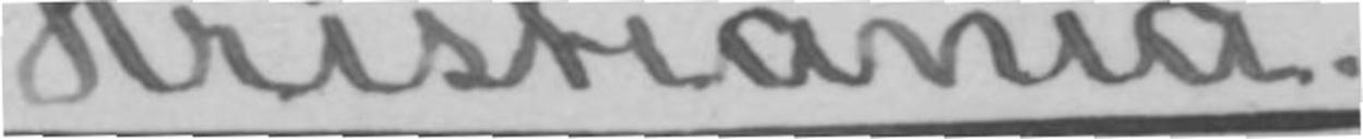Kristiania.
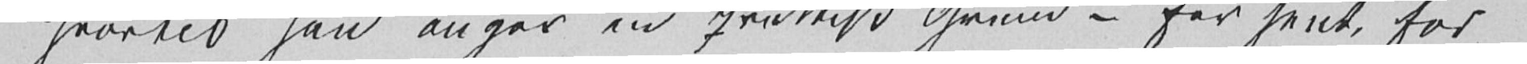hvortil han angav en praktisk Grund – for sent, for
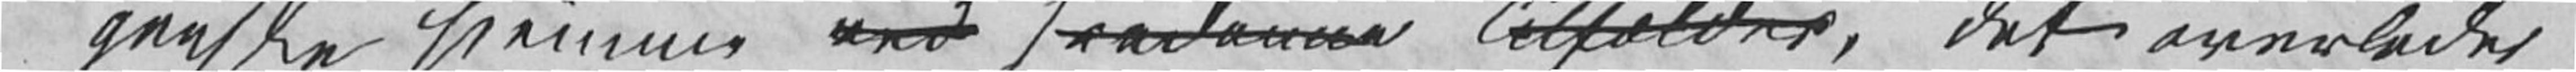ganske hjemme ved saadanne Tilfælder, det overlader
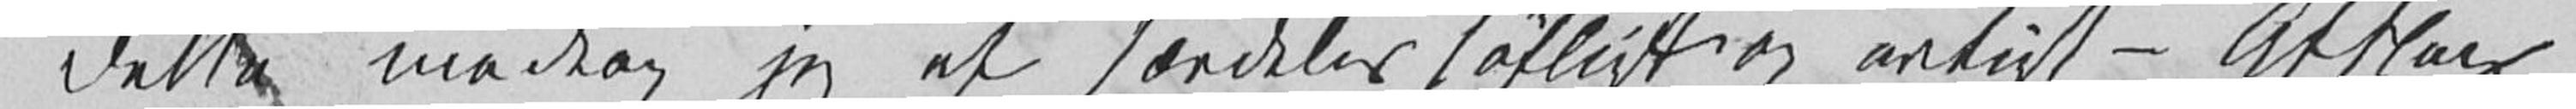dette modtog jeg et særdeles høfligt og artigt – Afslag
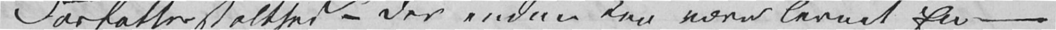Forfatterstolthet – der endnu kan være levnet En –
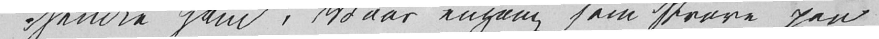sendte ham i Vaar engang som Prøve paa
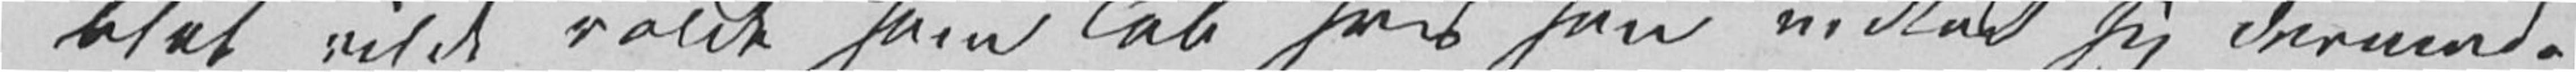blot vilde volde ham Tab hvis han indlod sig dermed.
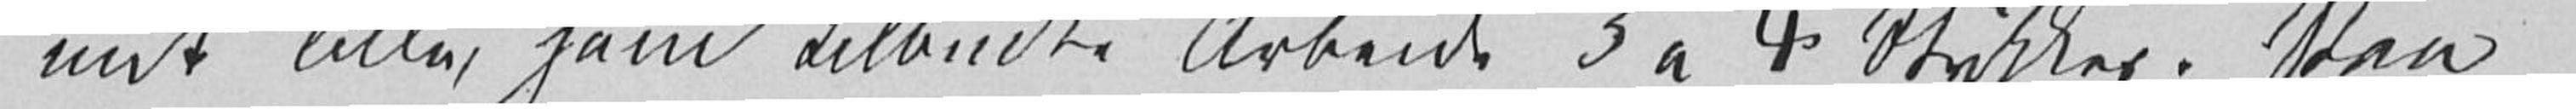mit lille, ham tilbudte Arbeide 3 a 4 Stykker. Paa
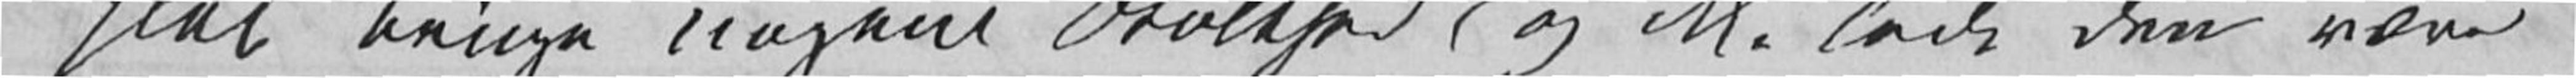skal bringe nogen Stolthed og ikke lade den være
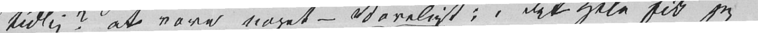tidlig? at vove noget – Voveligt: i det Hele fik jeg
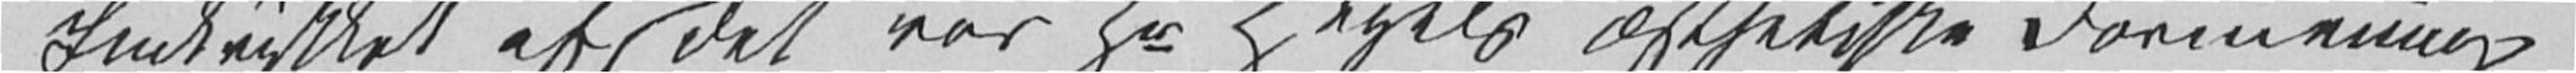Indtrykket af det var Hr Hegels æsthetiske Formening
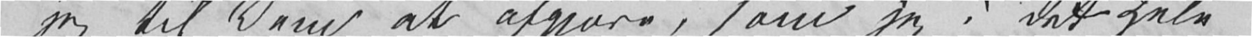jeg til Dem at afgjøre, som jeg i det Hele
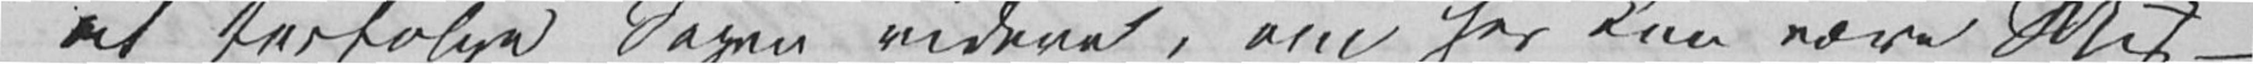at forfølge Sagen videre, om her kan være Mis¬
